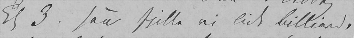Kl 3, saa spille vi lidt Billiard,
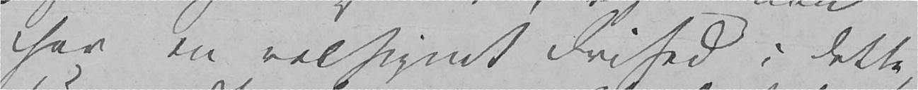har en velsignet Frihed i dette
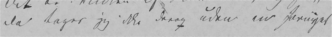da tager jeg ikke derop uden en fornyet
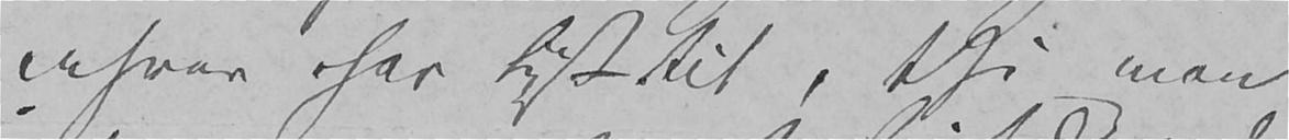enhver har Lyst til, thi man
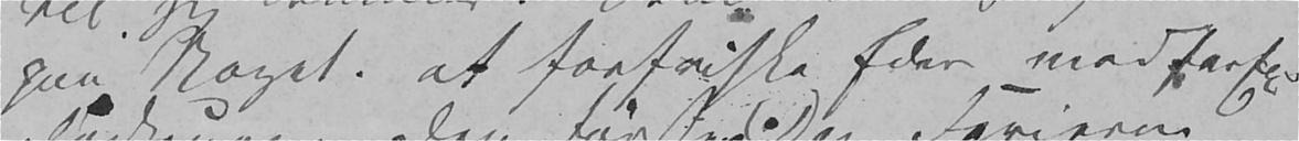paa Noget. at forfriske Eder med for Ex.
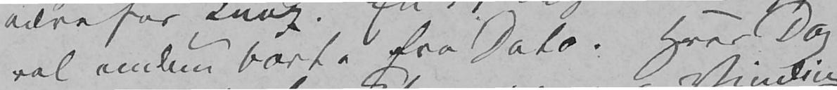vel endnu borte fra Dato. Hver Dag
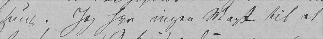Huus. Jeg har ingen Magt til at
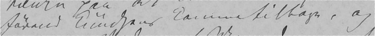førend Knudsens komme tilbage, og
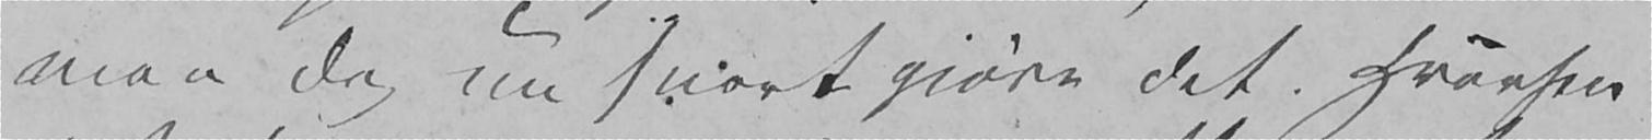maa dog nu snart gjøre det. Hvorhen
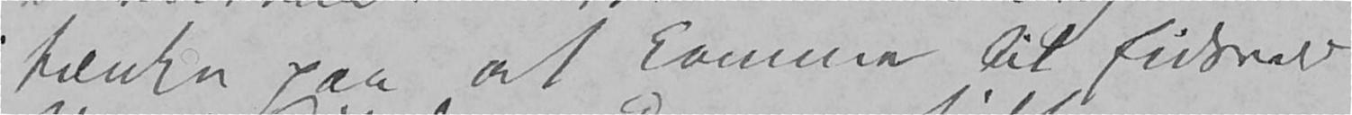tænke paa at komme til Eidsvold
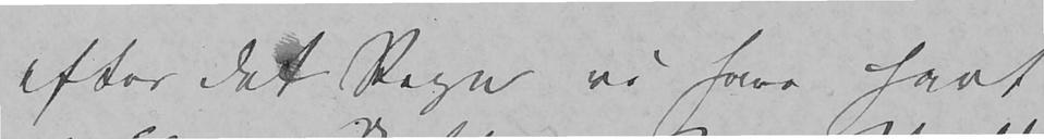efter det Regn vi have havt
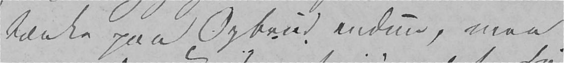tænke paa Opbrud endnu, men
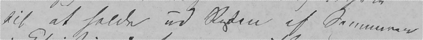til at holde ud Resten af Sommeren
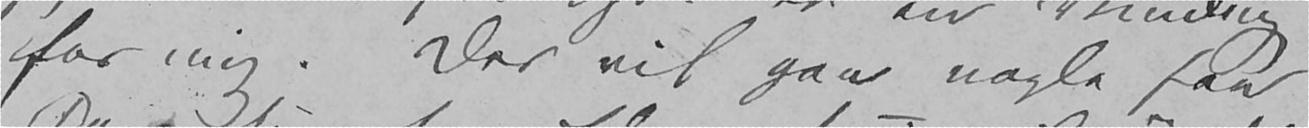for mig. Der vil gaa nogle faa
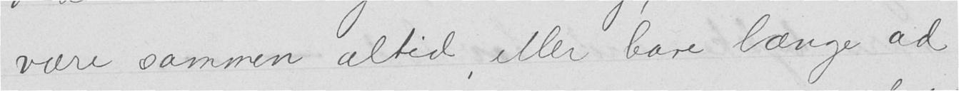være sammen altid, eller bare længe ad
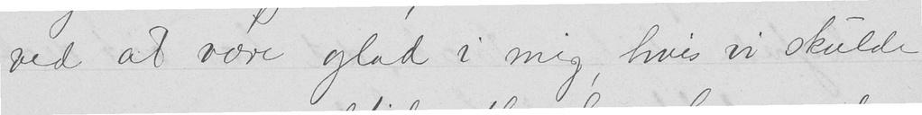ved at være glad i mig, hvis vi skulde
